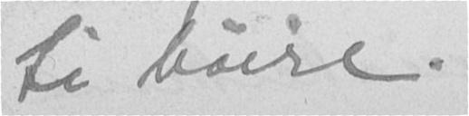ti høire.
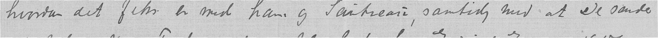hvordan det f eks. er med ham og Sautreau, samtidig med at De sender
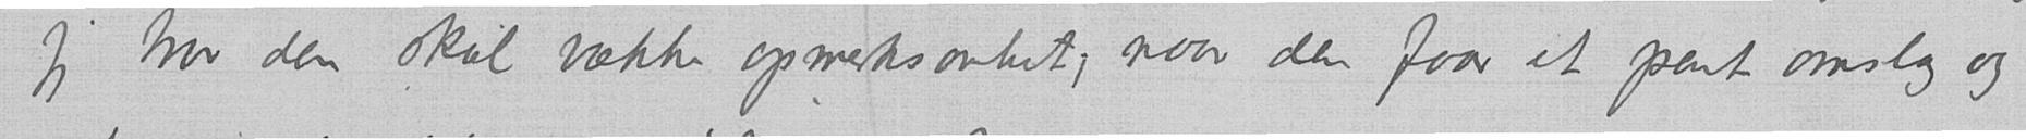jeg tror den skal vække opmerksomhet, naar den faar et pent omslag og
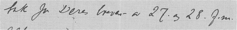tak for Deres brever  av 27. og 28. f.m.
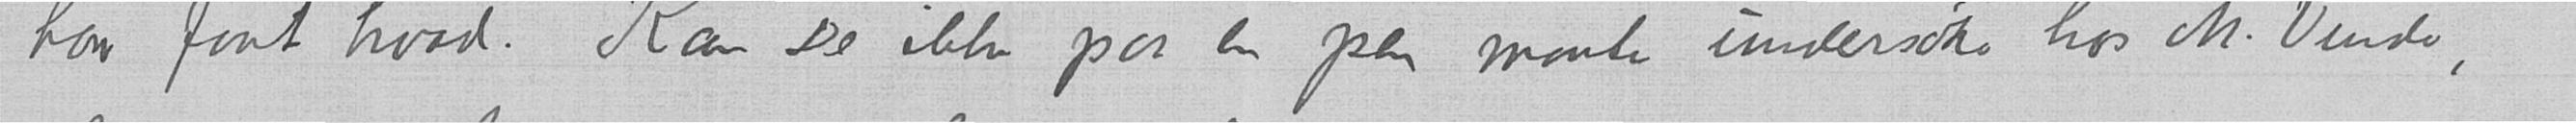har faat hvad. Kan De ikke paa en pen maate undersøke hos M. Vinde
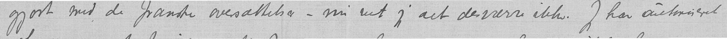gjort med de franske oversættelser – nu vet jeg det desværre ikke. Jeg har autoriseret
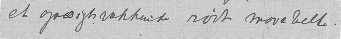et opsigtsvækkende rødt mavebelte.
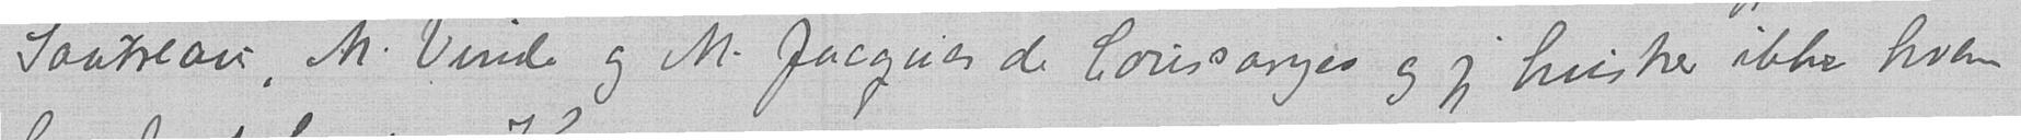Sautreau, M. Vinde og M. Jacques de Loussanges og jeg husker ikke hvem
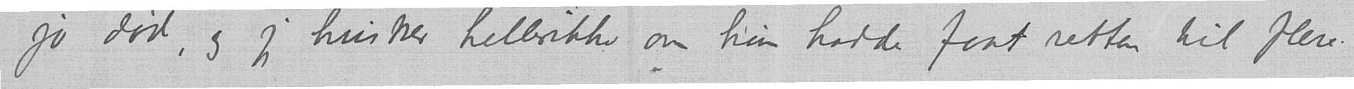jo død, og jeg husker hellerikke om hun hadde faat retten til flere.
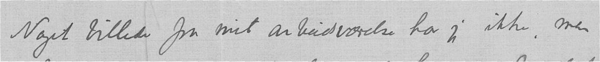Noget billede fra mit anbeidsværelse har jeg ikke, men
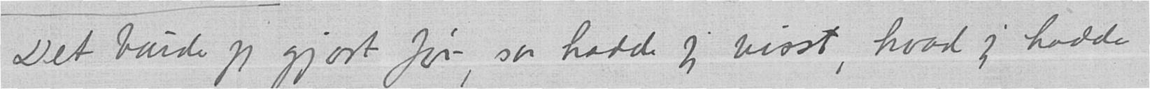Det burde jeg gjort før, saa hadde jeg visst, hvad jeg hadde
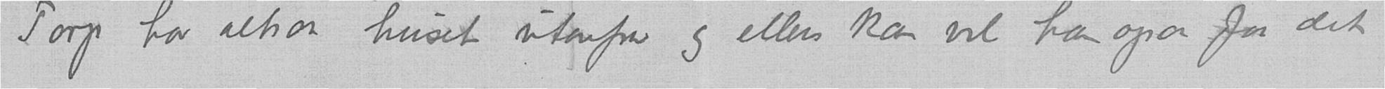Torp har altsaa huset utenfra og ellers kan vel han ogsaa faa det
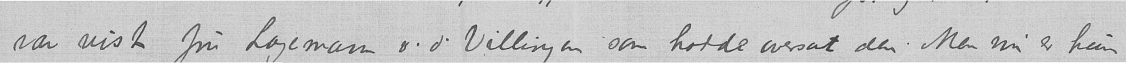var vist fru Lagemann v.d. Villingen som hadde oversat den. Men nu er hun
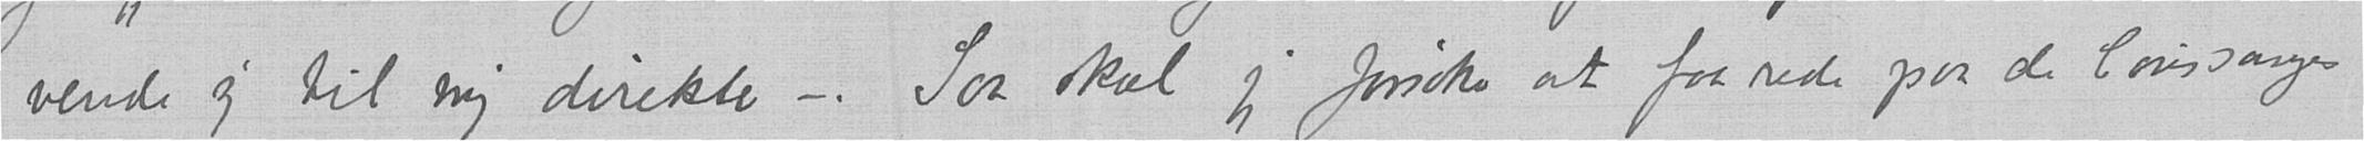vende sig til mig direkte –. Saa skal jeg forsøke at faa rede paa de Loussanges
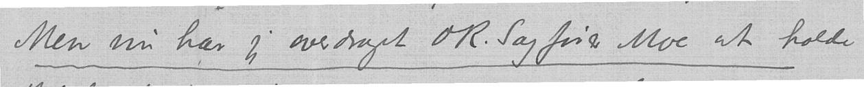Men nu har jeg overdraget OR. Sagfører Moe at holde
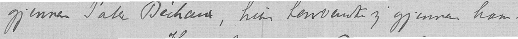gjennem Pater Bichaux, hun henvendte sig gjennem ham-.
